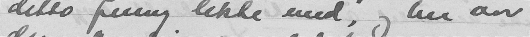ditto freng lekte med, og her var
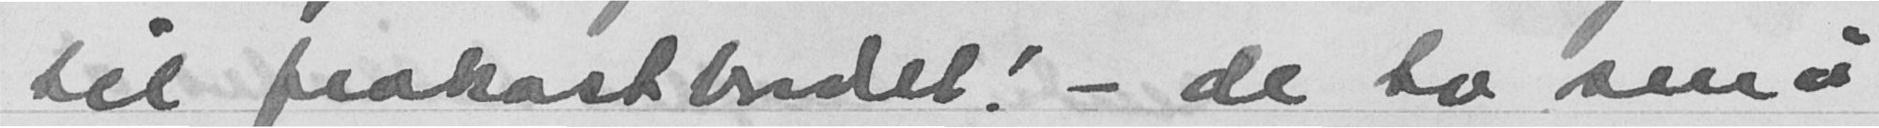til frokostbordet - de to små
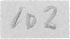102
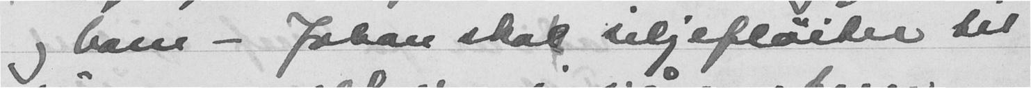og barn - Johan skar seljefløiter til
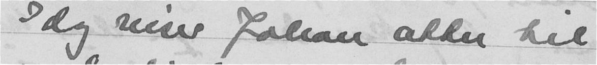I dag reiser Johan atter til
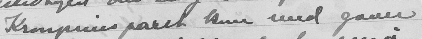Kronprinsparet kom med gaver
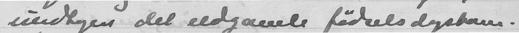undtagen det eldgamle fødselsdagsbarn.
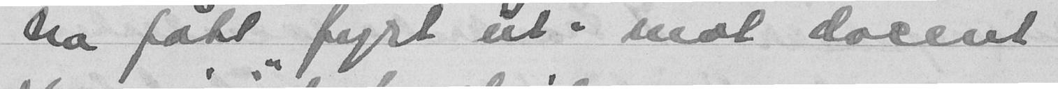nå fått fyrt ut mot docent
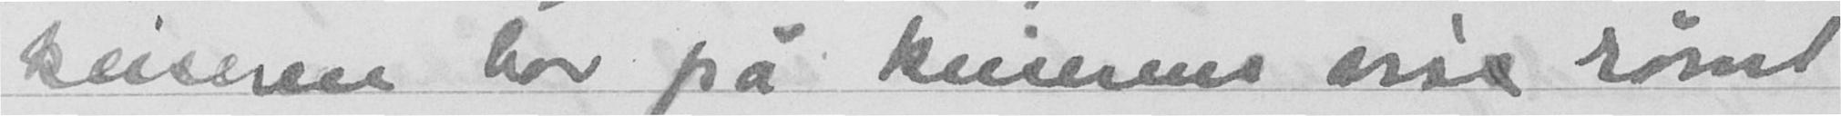keiseren har på keiserens vis rømt
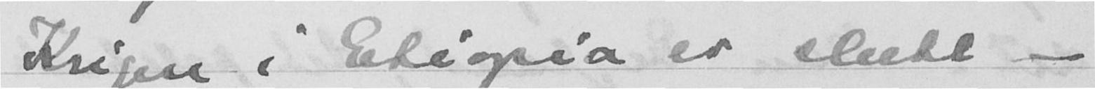Krigen i Etiopia er slutt -
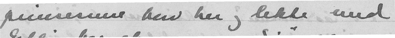pinsessene blev her og lekte med
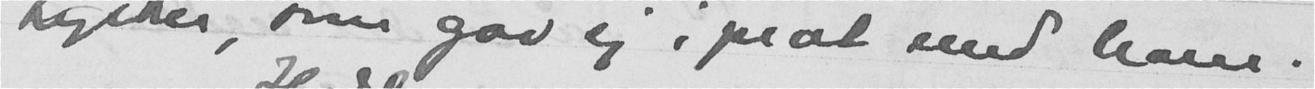tysker, som gav sig i prat med ham.
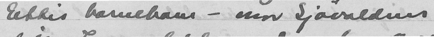Ellis barnebarn - mor Sjøvoldem
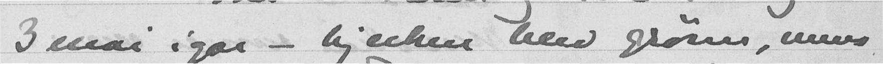3 mai i går - bjerken ble grønn, mens
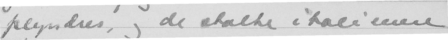plyndres, og de stolte italenere
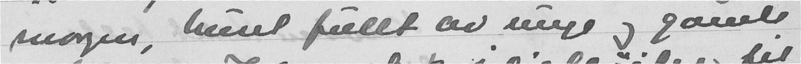morgen, huset fullt av unge og gamle,
